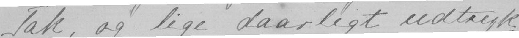Tak, og lige daarligt udtryk-
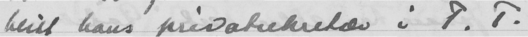blitt hans privatsekretær i F. T.
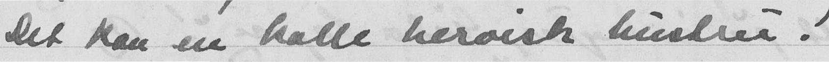Det kan en kalle heroisk hustru!
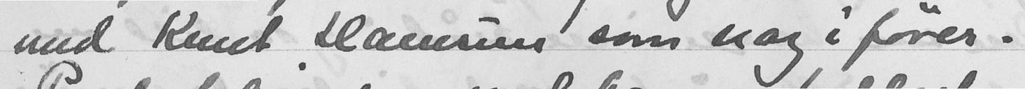med Knut Hamsun som svag i fører.
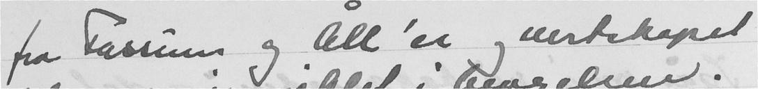fra Fossum og Ålt'er og vertskpet
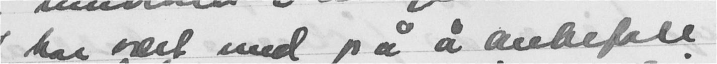Jeg har vært med på å anbefale
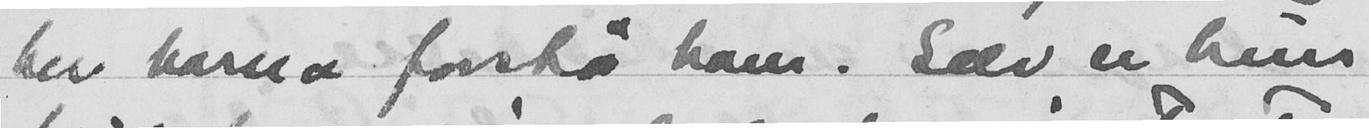lar barna forstå ham. Selv er hun
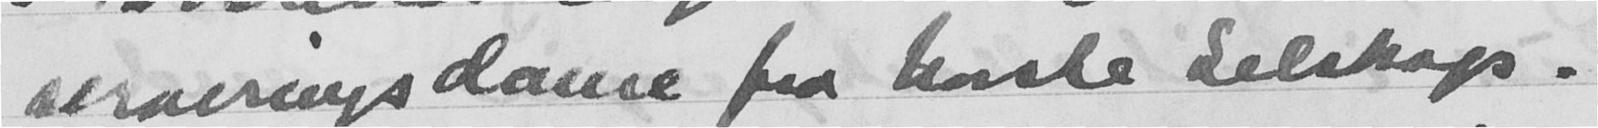serveringsdame fra beste Selskap.
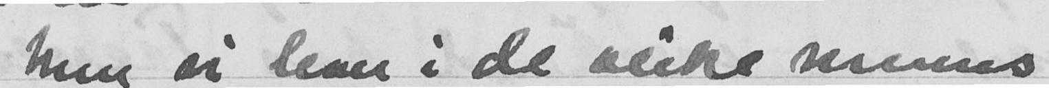Men vi lever i de slike menns
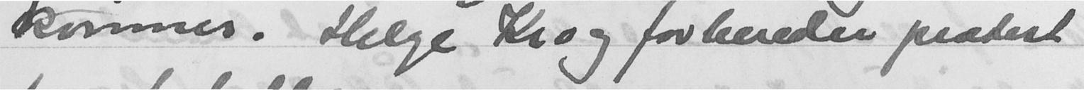kvinner. Helge Krog forbereder protest
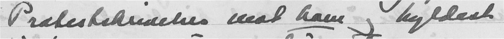Protestskrivelser mot ham og hyldest
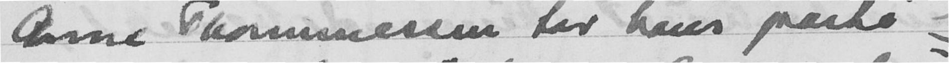Anne Thommessen tar hans parti og
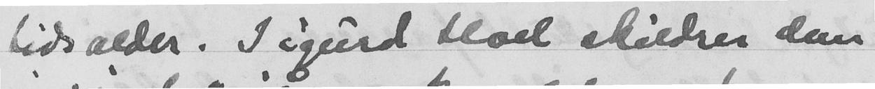tidsalder. Sigurd Hoel skildrer dem
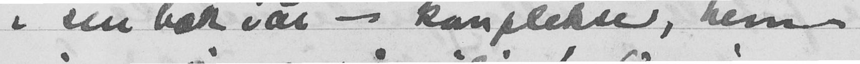i sin bok iår - komplekser, hevn
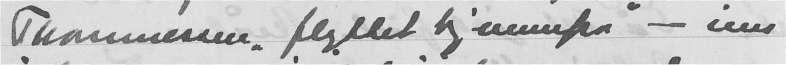Thommessen flyttet hjemmpå - inn
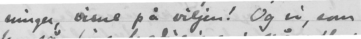smiger, visne på viljen. Og vi, som
